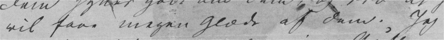vil faae megen Glæde af dem. Jeg
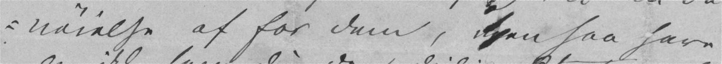-nøielse af for dem, men saa have
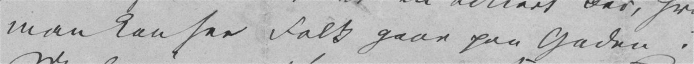man kan see Folk gaae paa Gaden i
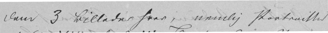dem 3 Billeder hver, nemlig Portraitter
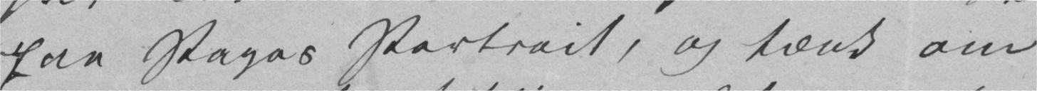paa Papas Portrait, og tænk om
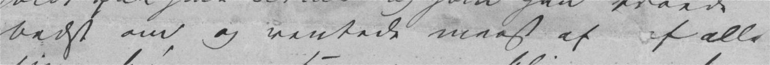bedst om og ventede meest af af alle
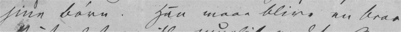sine Børn. Han maae blive en brav
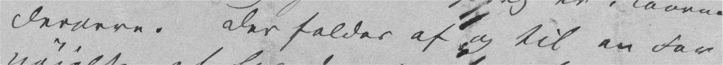derovre. Der falder af og til en For-
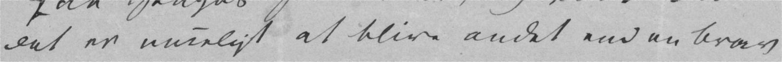det er mueligt at blive andet end en brav
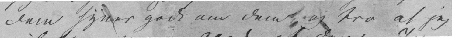dem synes godt om dem, og tro at jeg
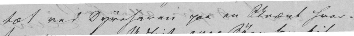tæt ved Dyrehaven paa en Skrænt hvor-
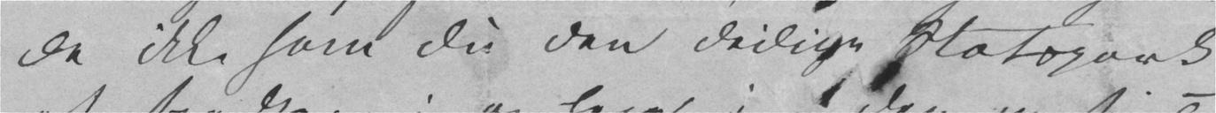de ikke som Du den deilige Slotspark
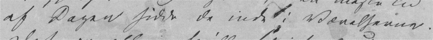af Dagen sidde de inde i Værelserne.
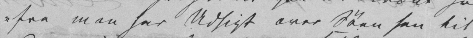fra man har Udsigt over Søen hen til
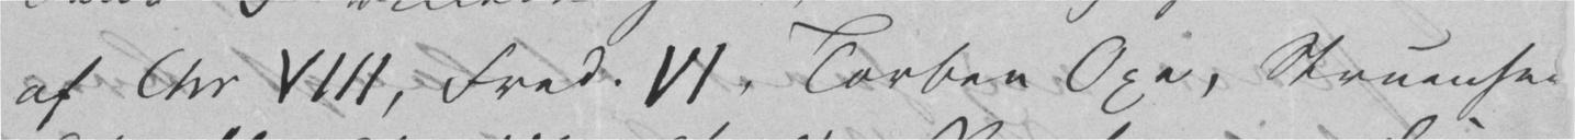af Chr VIII, Fred. VI, Torben Oxe, Struensee
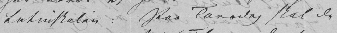Latinskolen. Paa Torsdag skal de
